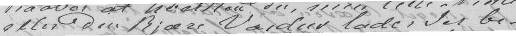eller Din kjære Vardus lader Jer be-
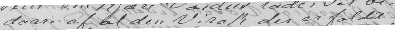daare af al den Virak der er faldet
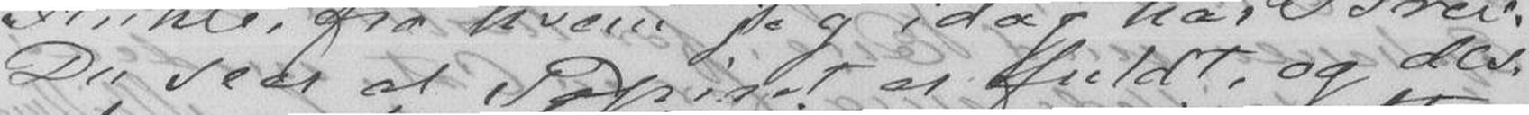Du seer at Papiret er fuldt, og des-
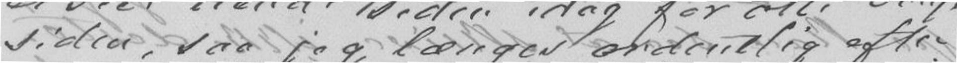siden, saa jeg længes ordentlig efter
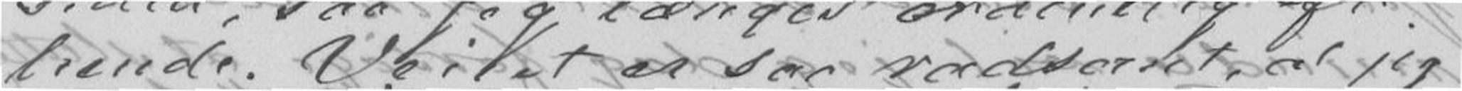hende. Veiret er saa radserrit, at jeg
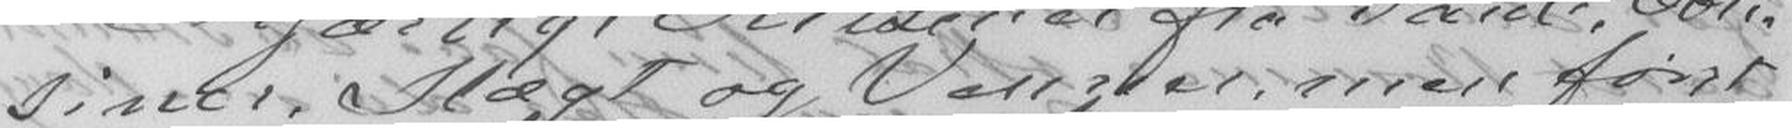siner, Slægt og Venner, men først
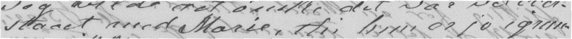staaet med Marie, thi hun er jo igrun-
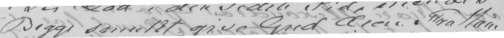Begge smukt give Gud Æren. Fra Ham
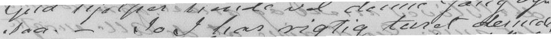saa. - Jo I har rigtig turet dernede
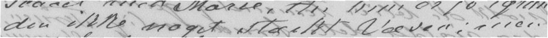den ikke noget stærkt Væsen; men
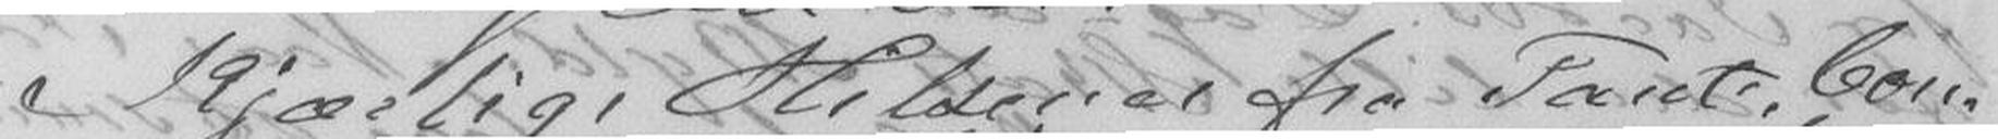Kjærlig, Hilsener fra Tante, Cou-
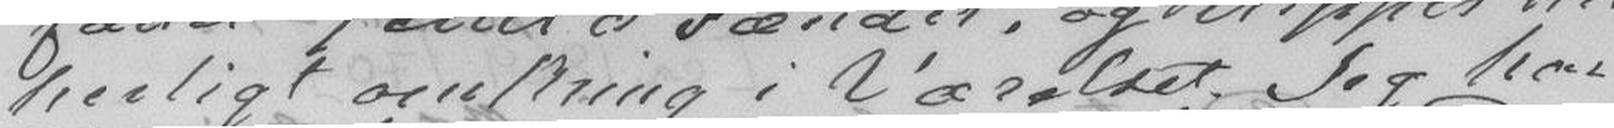herligt omkring i Værelset. Jeg har
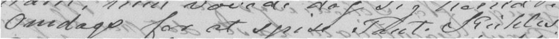Onsdags for at spise Tante Kühles
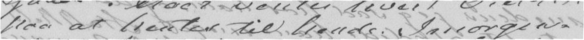Gaas. Moer ventes til hende. Imorgen-
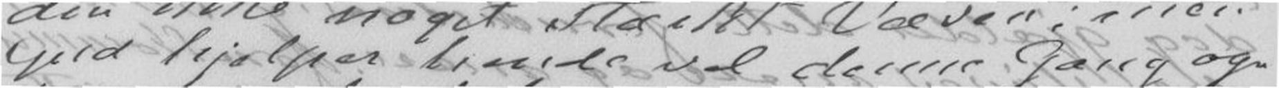Gud hjelper hande vel denne Gang og-
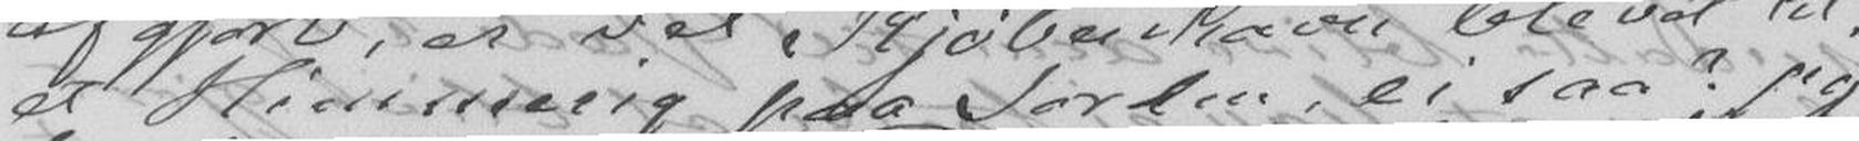et Himmerig paa Jorden, ei saa? jeg
